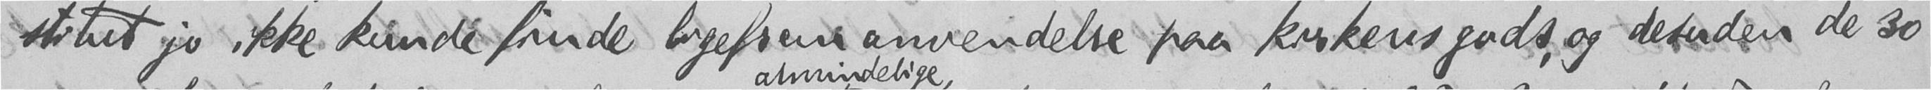stitut jo ikke kunde finde ligefrem anvendelse paa kirkens gods, og desuden de 30
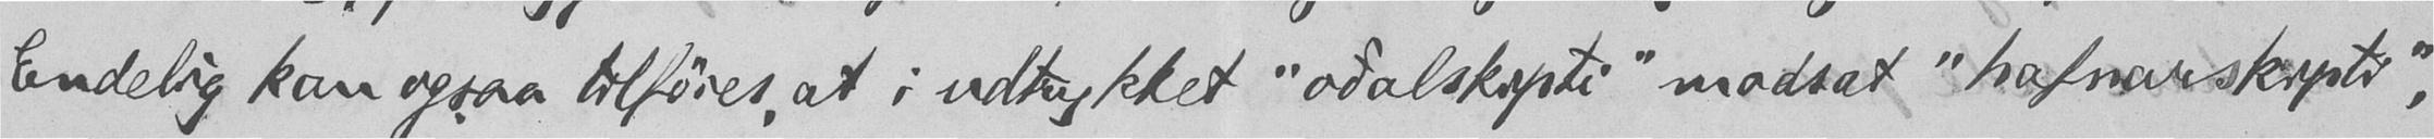Endelig kan ogsaa tilføies, at i udtrykket "oðalskipti" modsat "hafnarskipti",
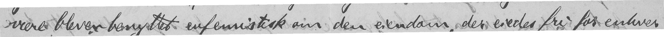være bleven benyttet eufemistisk om den eiendom, der eiedes fri for enhver
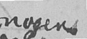nogens
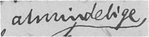almindelige
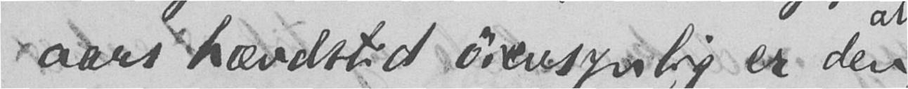aars hævdstid øiensynlig er den
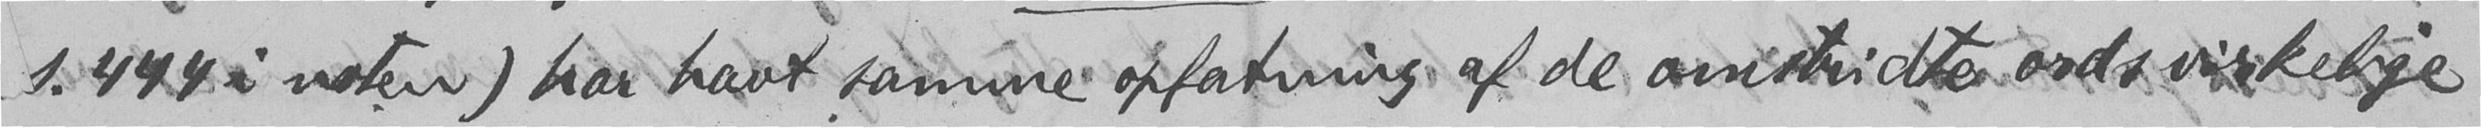s. 444 i noten) har havt samme opfatning af de omstridte ords virkelige
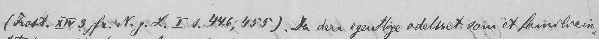(Frost. XIV 3 jfr. N. g. L. I s. 446, 455). Da den egentlige odelsret som et familiein-
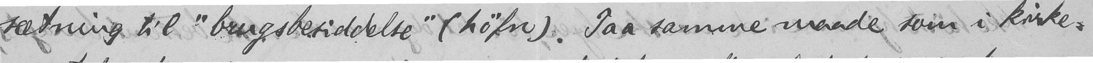sætning til "brugsbesiddelse" (høfn). Paa samme maade som i kirke-
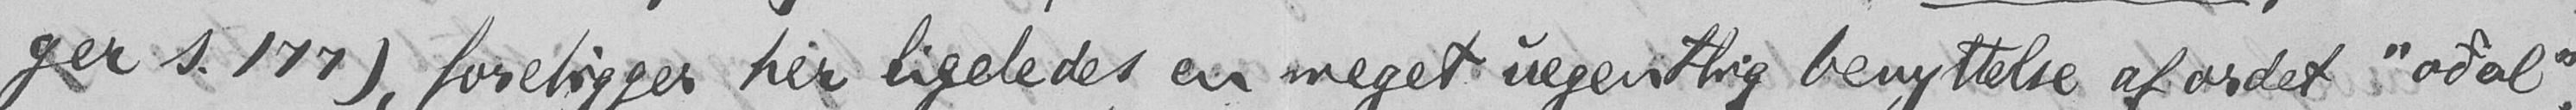ger s. 177), foreligger her ligeledes en meget uegentlig benyttelse af ordet "oðal".
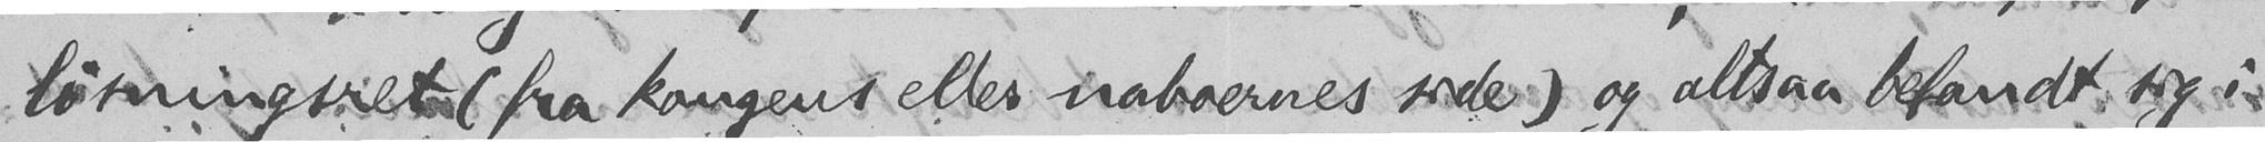løsningsret (fra kongens eller naboernes side) og altsaa befandt sig i
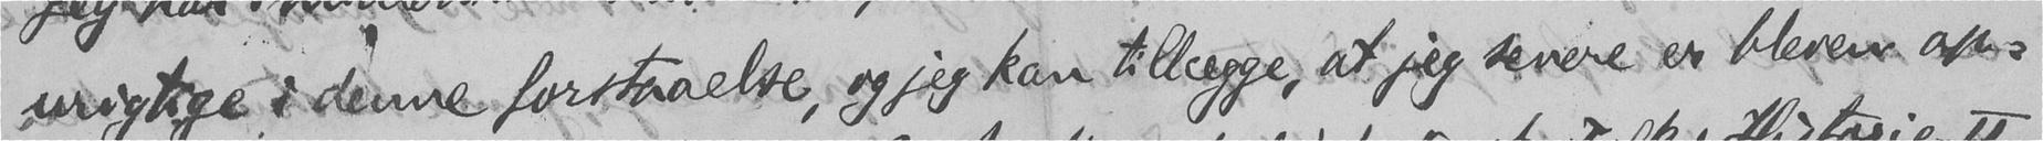urigtige i denne forstaaelse, og jeg kan tillægge, at jeg senere er bleven op-
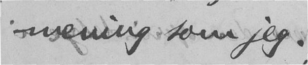mening som jeg.
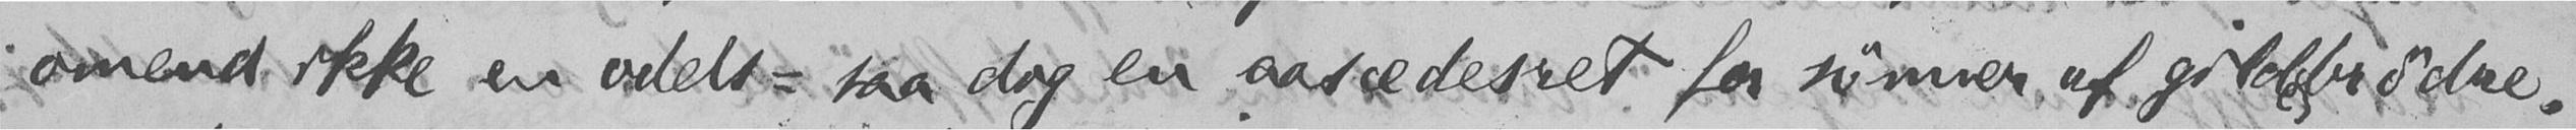omend ikke en odels- saa dog en aasædesret for sønner af gildebrødre.
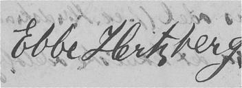Ebbe Hertzberg
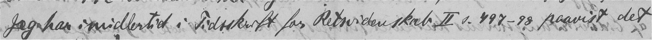Jeg har imidlertid i Tidsskrift for Retsvidenskab II s. 497-98 paavist det
